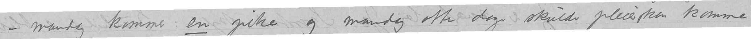mandag kommer en pike og mandag etter deg skulde pleiersken komme
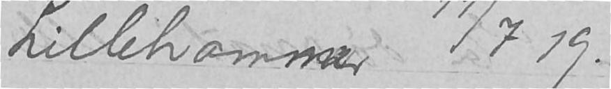Lillehammer 11/7 19.
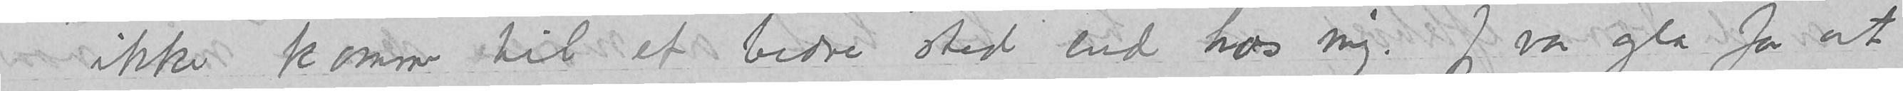ikke komme til et bedre sted end hos mig. Jeg var gla for at
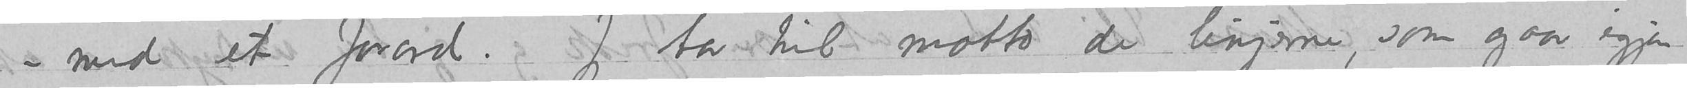- med et forord. Jeg tar til motto de linjerne, som gaar igjen
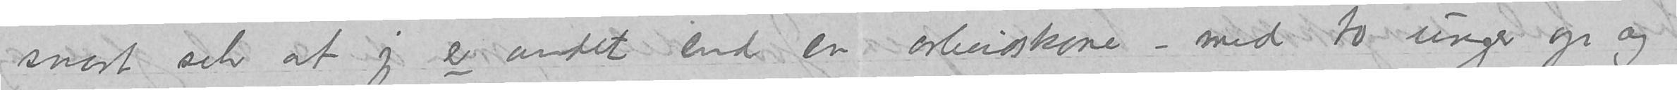snart selv at jeg er andet end en arbeidskone – med to unger op og
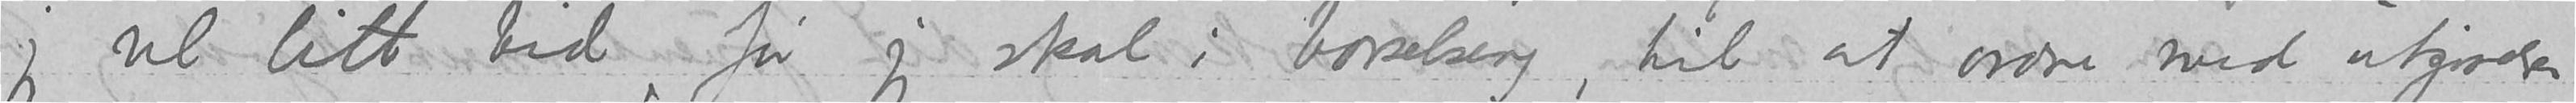jeg vel litt tid før jeg skal i barselseng, til at ordne med utgivelsen
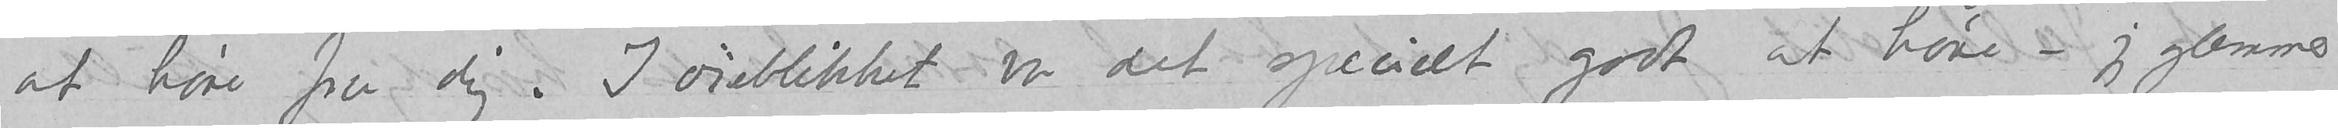at høre fra dig. I øieblikket var det specielt godt at høre – jeg glemmer
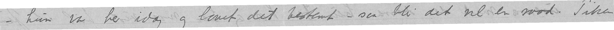hun var her idag og lovet det bestemt – saa blir det vel en raad. Piken
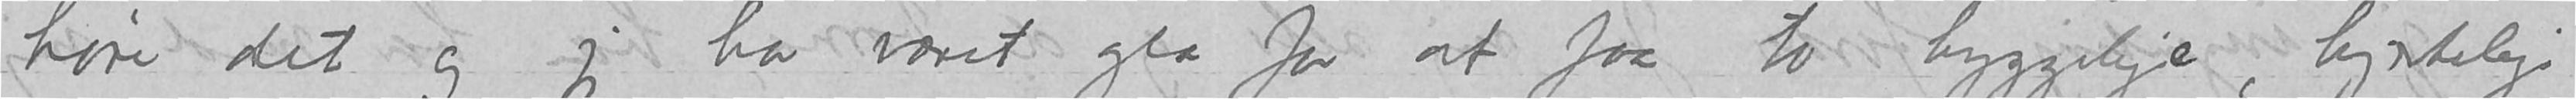høre det og jeg har været gla for at faa to hyggelige, hjertelige
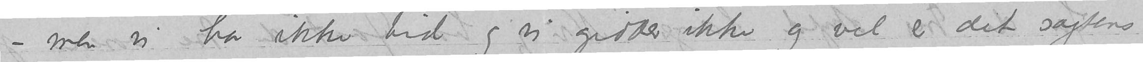men vi har ikke tid og vi gidder ikke og vel er det sagtens
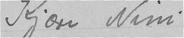Kjære Nini
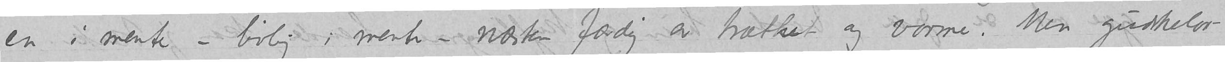en i mente – livlig i mente – næsten færdig av træthet og varme. Men gudskelov
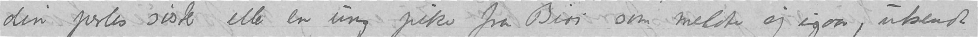din perles søster eller en ung pike fra Biri som meldte sig igaar, utsendt
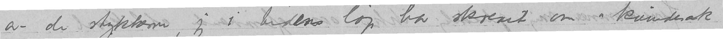av de stykkerne, jeg i tidens løp har skrevet om «kvindesak»
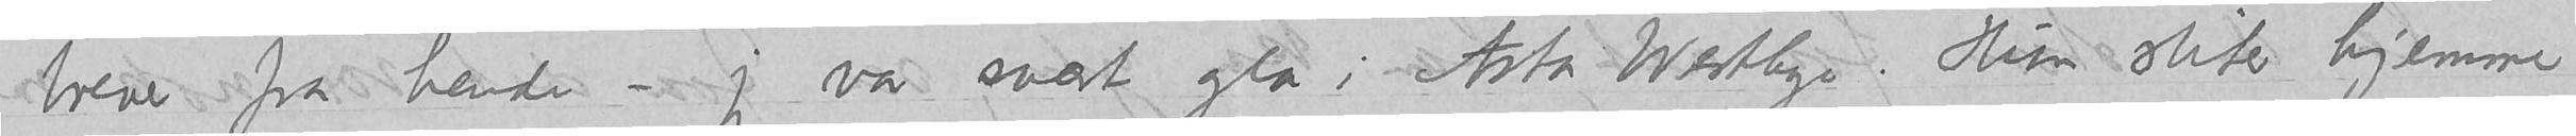brever fra hende – jeg var svært gla i Asta Westbye. Hun sliter hjemme
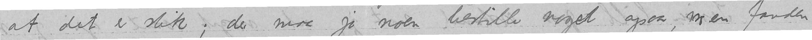at det er slik; der maa jo noen bestille noget ogsaa, men fanden
